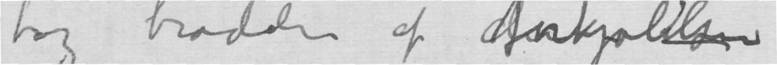tog brodden af forkjølelsen
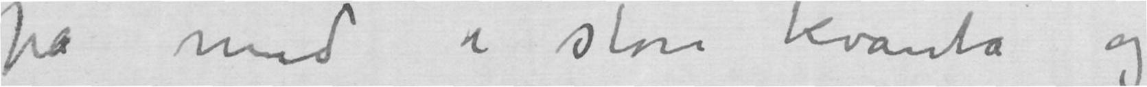på med i store kvanta og
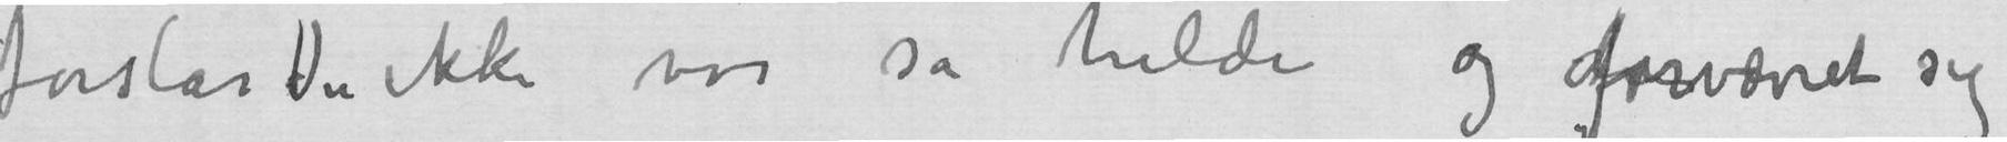forstår Du ikke var så heldi og forværret sig
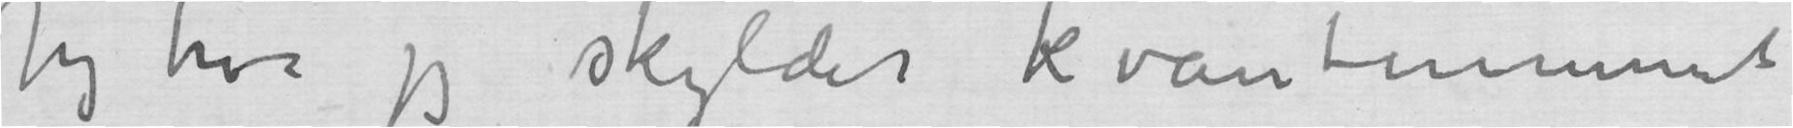Jeg tror jeg skylder kvantummet
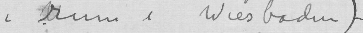i rum i Wiesbaden–)
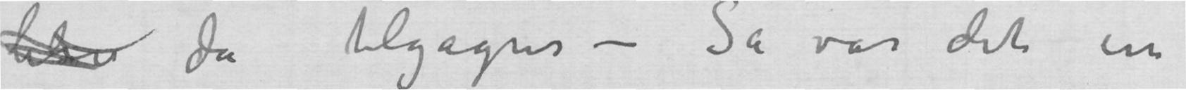blev da tilgagns – Så var det en
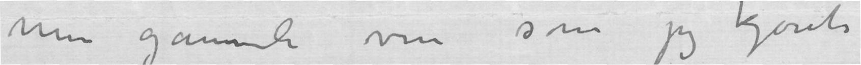min gamle ven som jeg kjørte
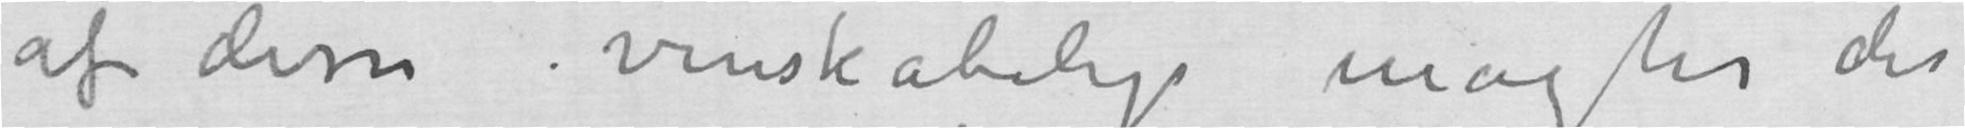af disse venskabelige magter der
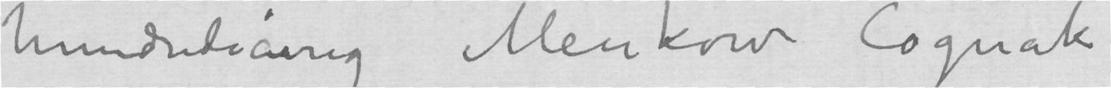hundreårig Menkow Cognak
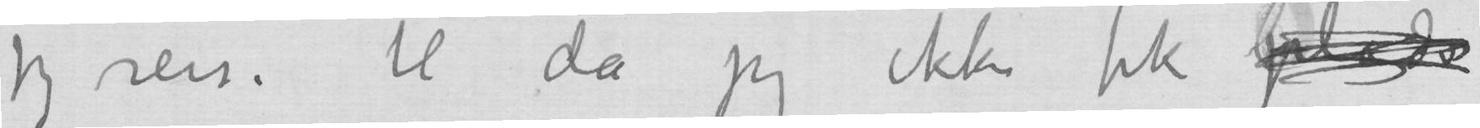jeg reise til da jeg ikke fik plads
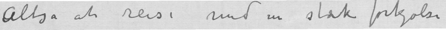Altså at reise med en stærk forkjølse
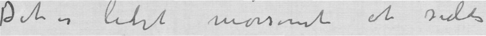Det er lidet morsomt at sidde
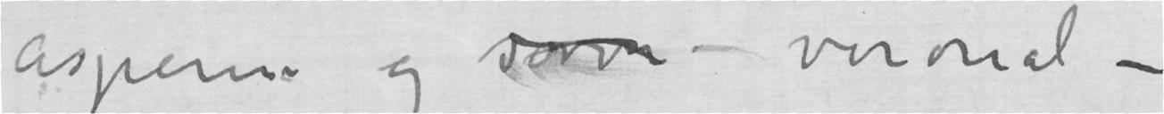aspirin og s veronal –
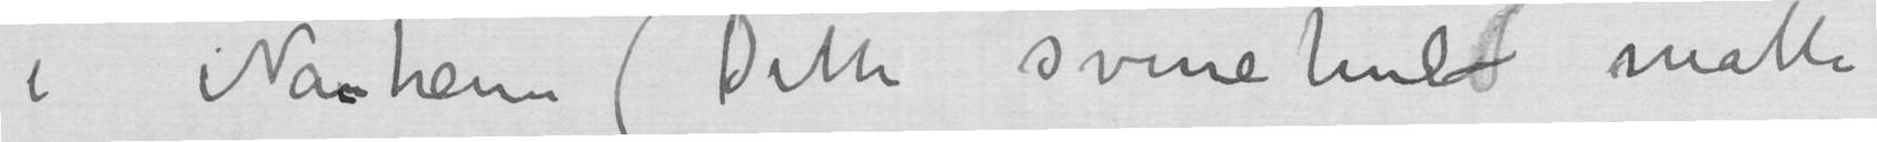i Nauheim (Dette svinehul måtte
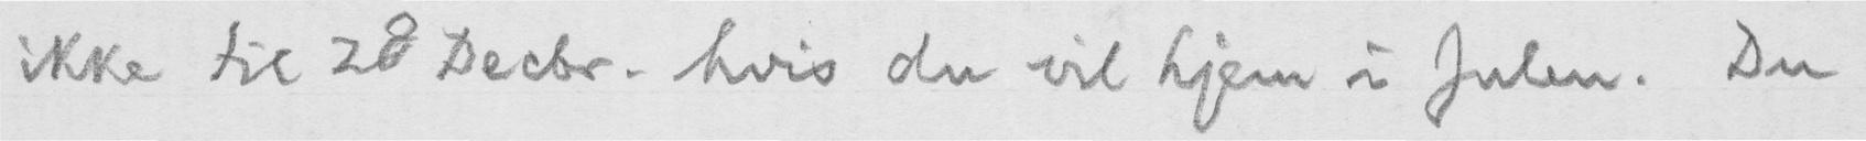ikke til 28 Decbr. hvis du vil hjem i Julen. Du
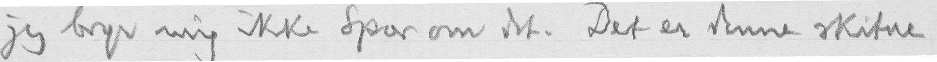jeg bryr mig ikke Spor om det. Det er denne skitne
